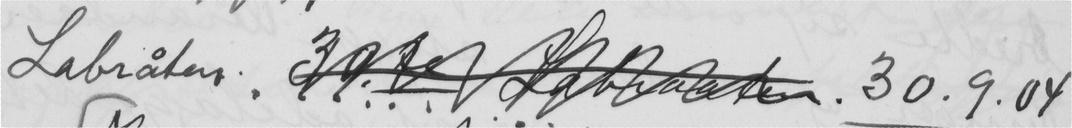Labråten.  30.9.04
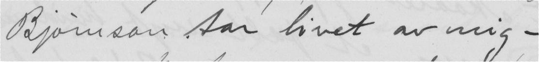Bjørnson tar livet av mig -
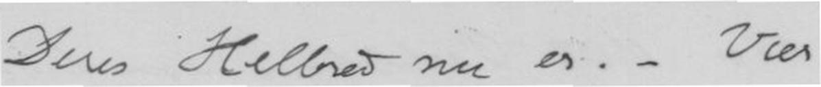Deres Helbred nu er. - Vær
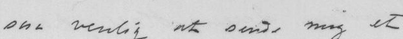saa venlig at sende mig et
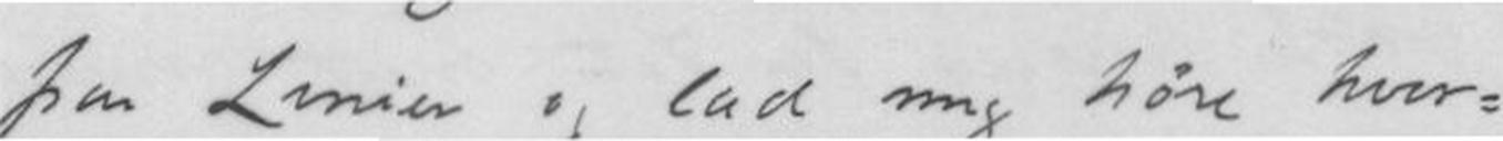par Linier og lad mig høre hvor-
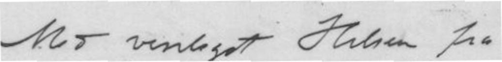Med venligst Hilsen fra
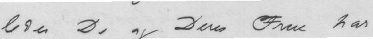ledes De og Deres Frue har
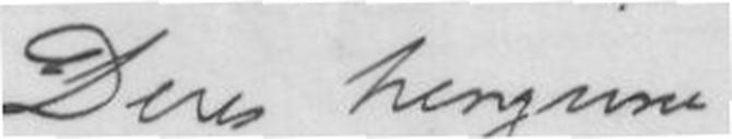Deres hengivne
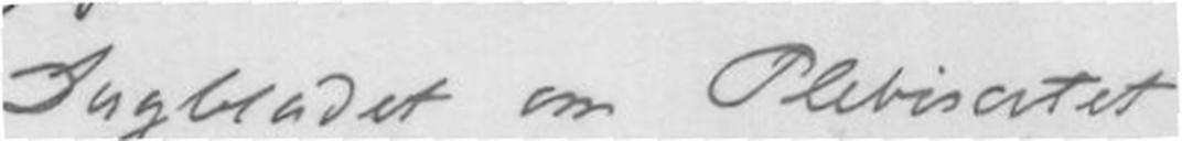Dagbladet om Plebiscitet!
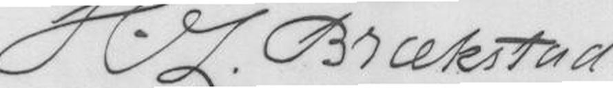H.L. Brækstad
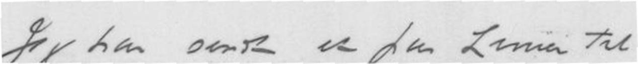Jeg har sendt et par Linier til
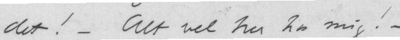det! - Alt vel her hos mig! -
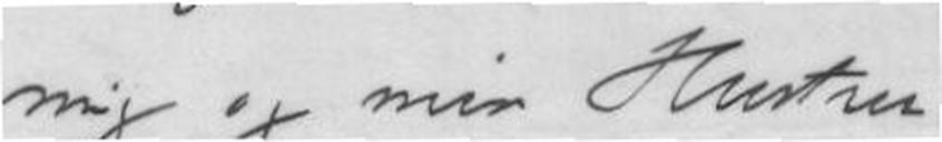mig og min Hustru
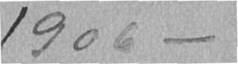1906 -
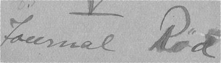Journal Rød
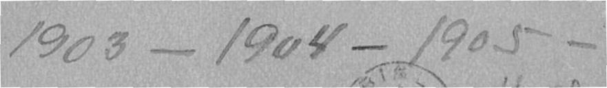1903 - 1904 - 1905 -
